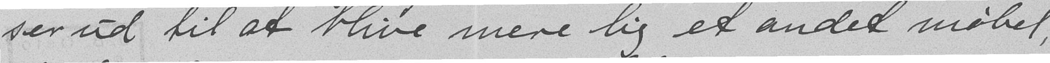ser ud til at blive mere lig et andet møbel,
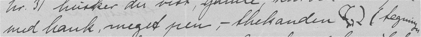med hank, meget pen, - thekanden (tegningen
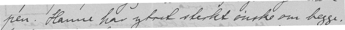pen. Hanne har ytret sterkt ønske om begge.
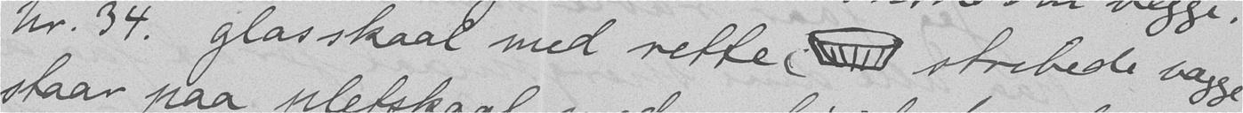Nr. 34. glasskaal med rette stribede vagge
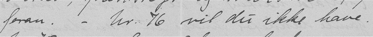foran. - Nr. 76 vil du ikke have.
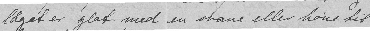låget er glat med en svane eller høne til
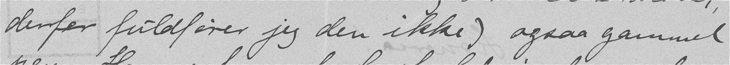derfor fuldfører jeg den ikke) ogsaa gammel
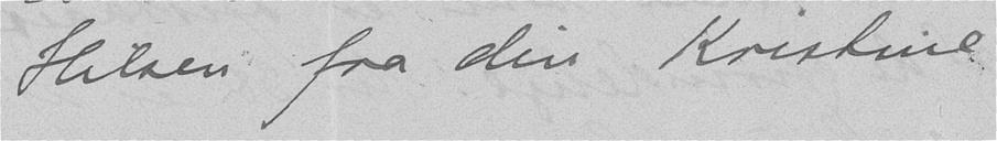Hilsen fra din Kristine
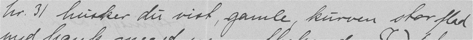Nr. 31 husker du vist, gamle, kurven stor flad
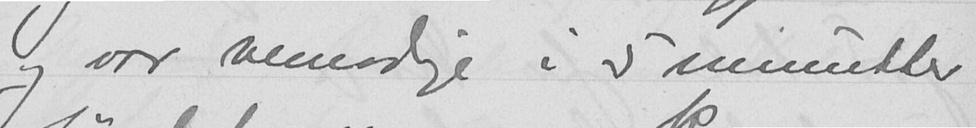og var vemodige i 5 minutter,
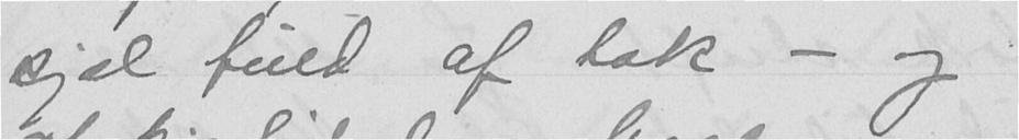sjæl fuld af tak - og
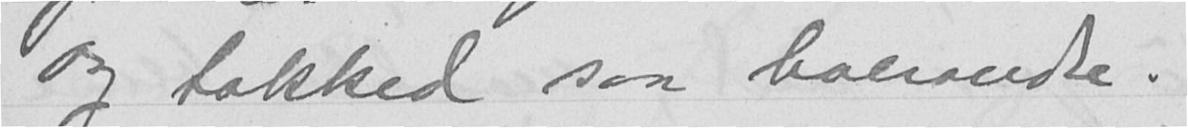Og takked saa hverandre.
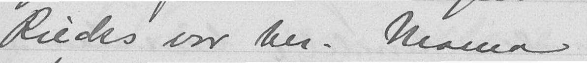Riecks var her. Mama
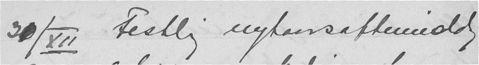31/XII Festlig nytaarsaftmiddag
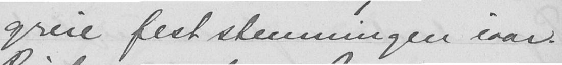greie feststemningen iaar.
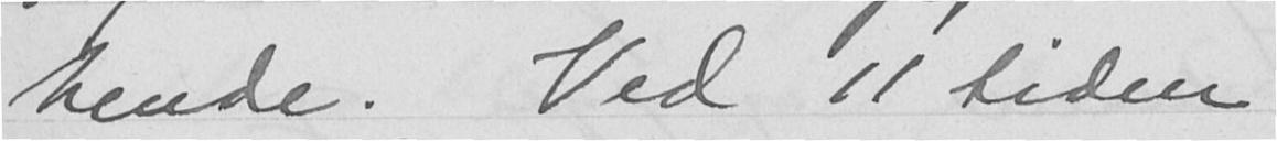hende. Ved 11 tiden
